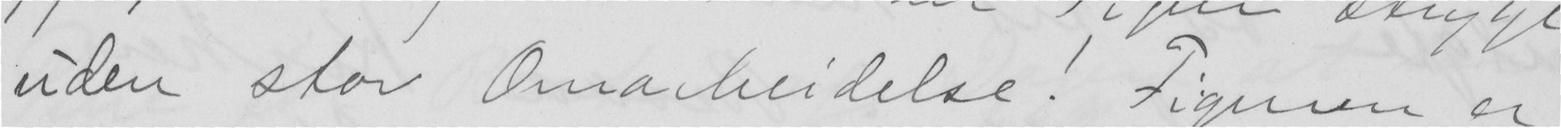uden stor Omarbeidelse! Figuren er
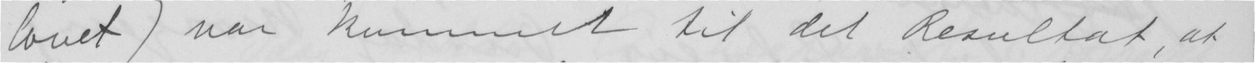lovet) var kommet til det Resultat, at
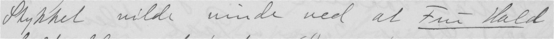Stykket vilde vinde ved at Fru Hald
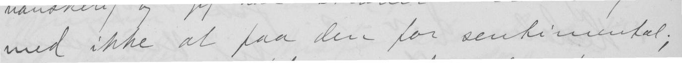med ikke at faa den for sentimental;
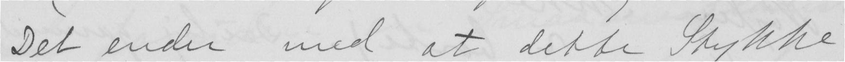Det ender med at dette Stykke
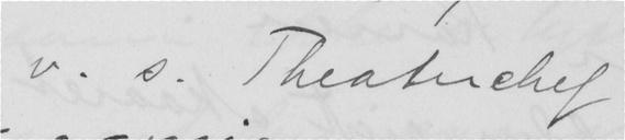v.s. Theaterchef
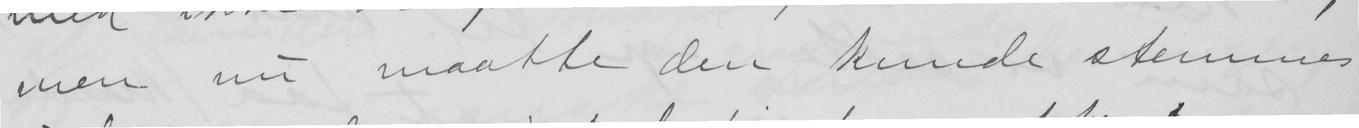men nu maatte den kunde stemmes
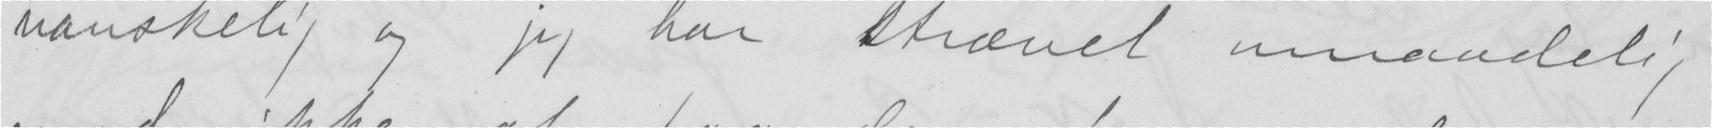vanskelig og jeg har strævet umaadelig
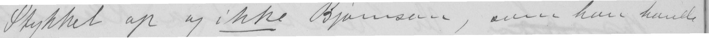Stykket op og ikke Bjørnson, som han havde
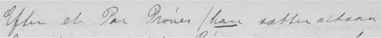Efter et Par Prøver (han sætter altsaa
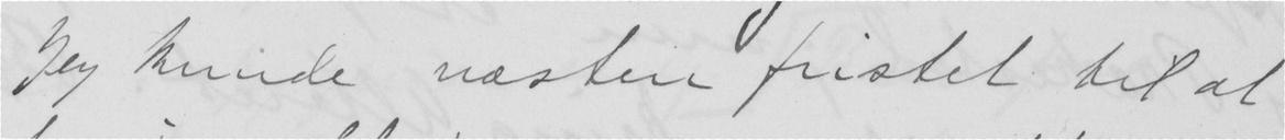Jeg kunde næsten fristet til at
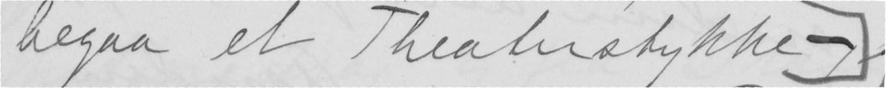begaa et Theaterstykke -
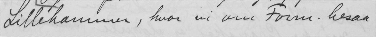Lillehammer, hvor vi om Form. besaa
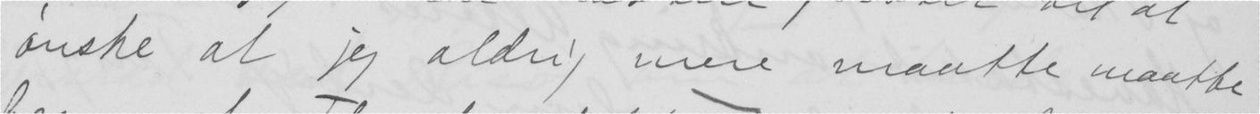ønske at jeg aldrig mere maatte maatte
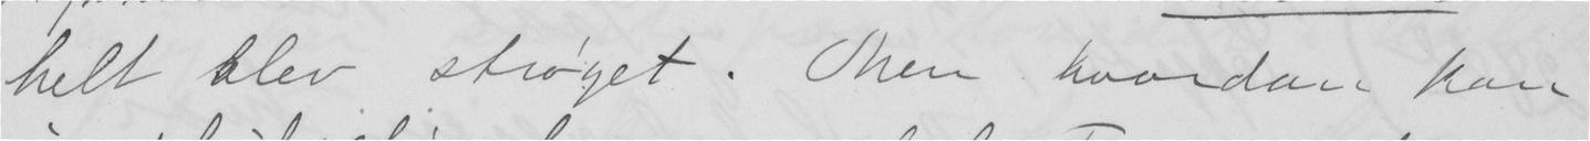helt blev strøget. Men hvordan kan
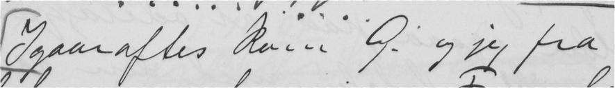Igaaraftes kom G. og jeg fra
